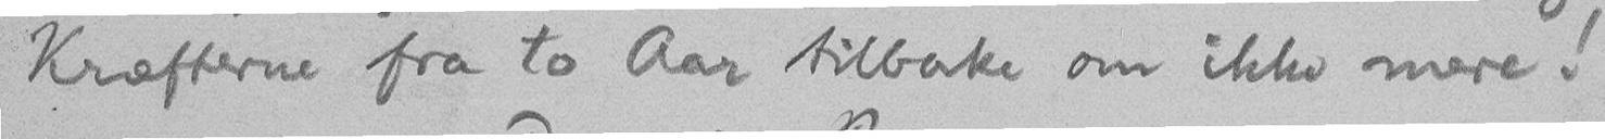Kræfterne fra to Aar tilbake om ikke mere!
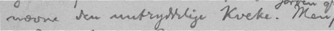nævne den uutryddelige Kveke. Men
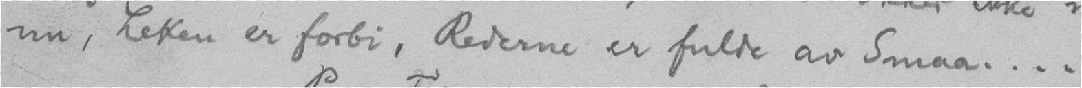nu, Leken er forbi, Rederne er fulde av Smaa....
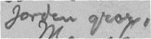Jorden gror,
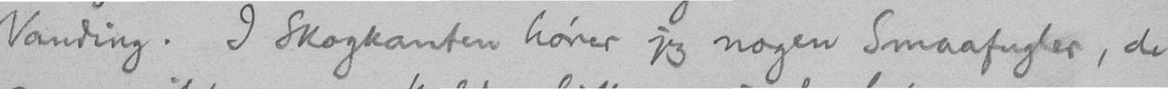Vanding. I Skogkanten hører jeg nogen Smaafugler, de
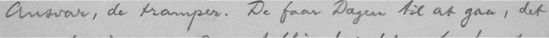Ansvar, de tramper. De faar Dagen til at gaa, det
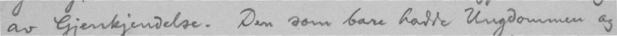av Gjenkjendelse. Den som bare havde Ungdommen og
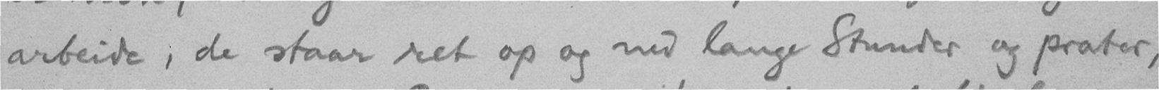arbeide, de staar ret op og ned lange Stunder og prater,
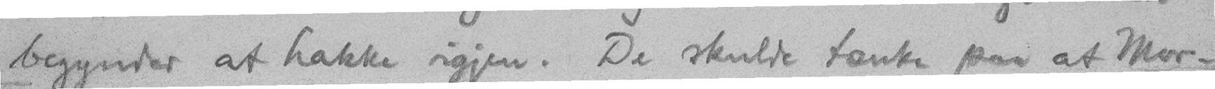begynder at hakke igjen. De skulde tænke paa at Mor-
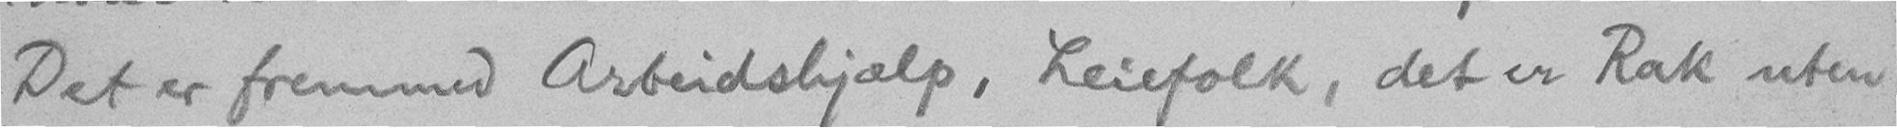Det er fremmed Arbeidshjælp, Leiefolk, det er Rak uten
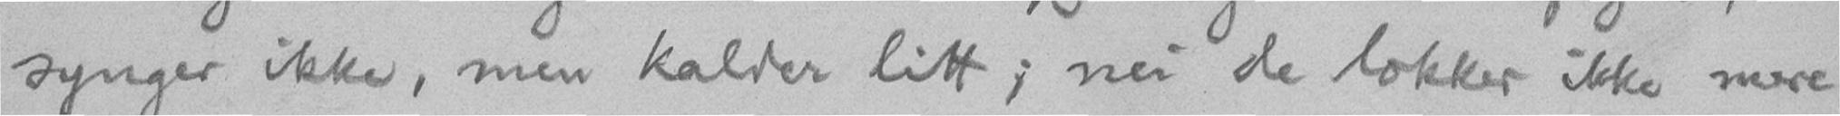synger ikke, men kalder litt; nei de lokker ikke mere
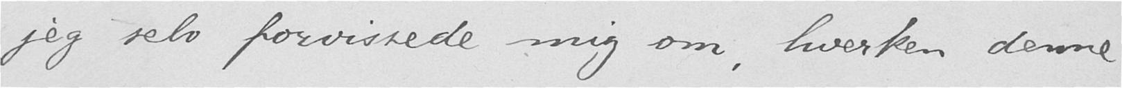jeg selv forvissede mig om, hverken denne
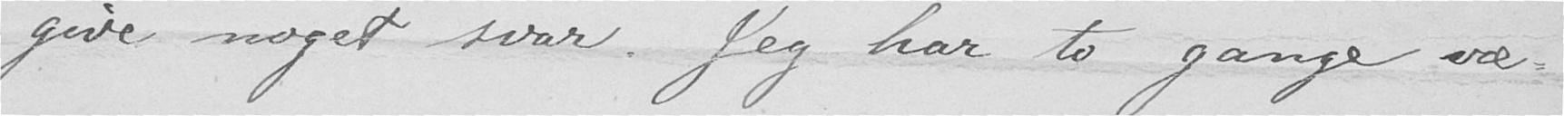give noget svar. Jeg har to gange væ-
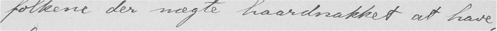folkene der nægte haardnakket at have,
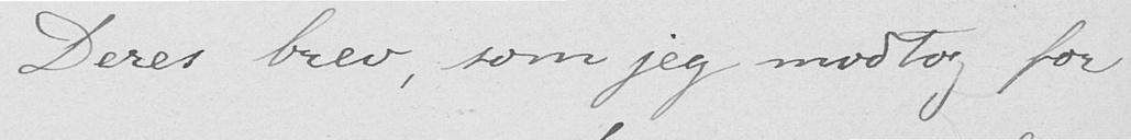Deres brev, som jeg modtog for
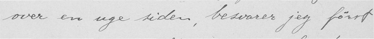over en uge siden, besvarer jeg først
